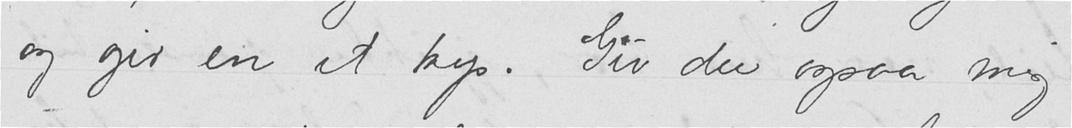og gir en et kys. Giv du ogsaa mig
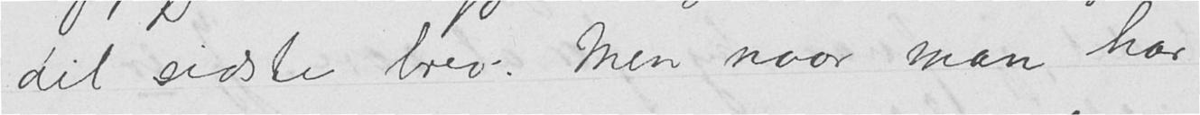dit sidste brev. Men naar man har
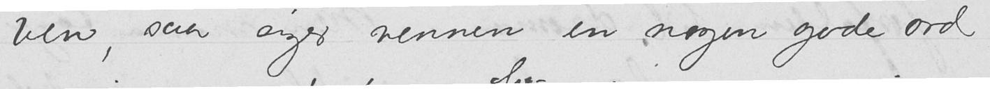ven, saa siger vennen en nogen gode ord
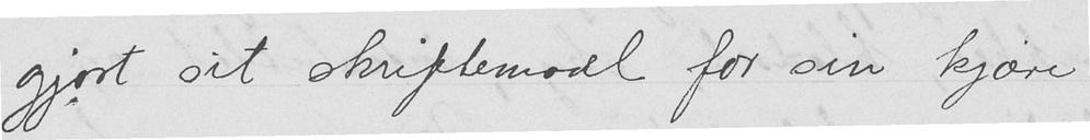gjort sit skriftemaal for sin kjære
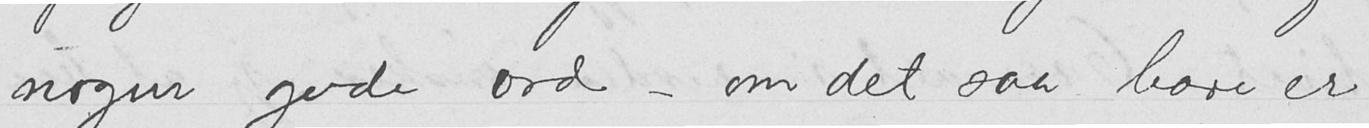nogen gode ord - om det saa bare er
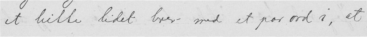et bitte lidet brev med et par ord i, at
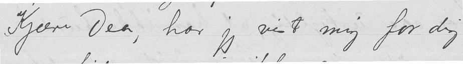Kjære Dea, har jeg vist mig for dig
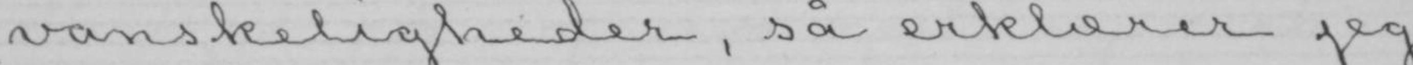vanskeligheder, så erklærer jeg
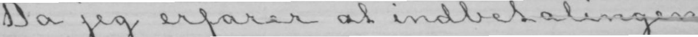Da jeg erfarer at indbetalingen
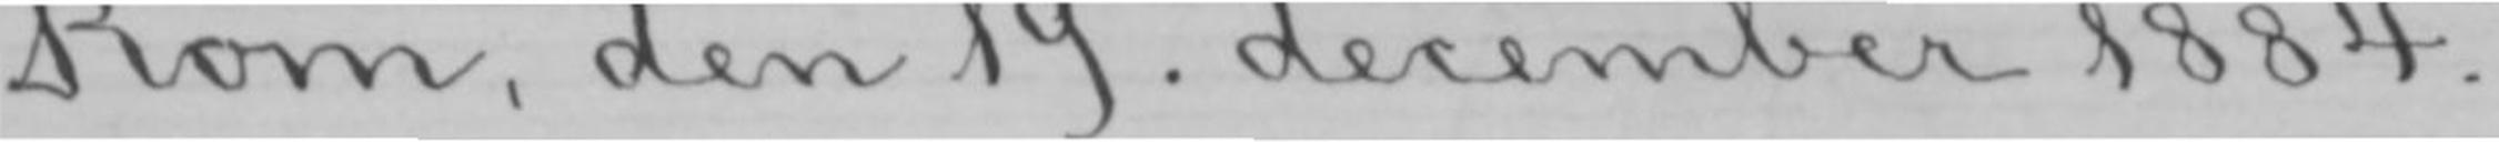Rom, den 19. december 1884.
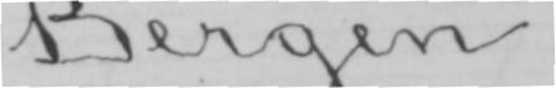Bergen.
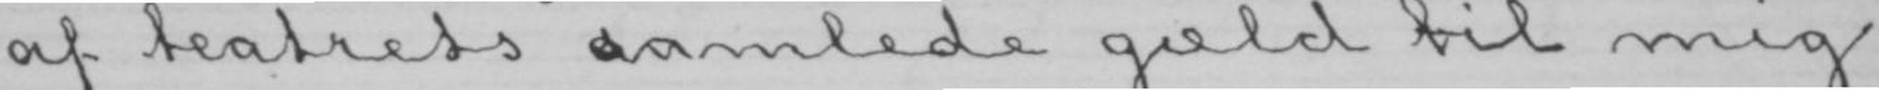af teatrets samlede gæld til mig
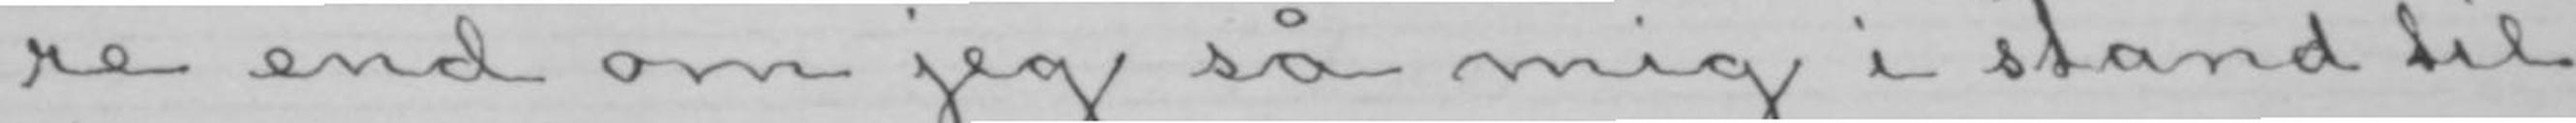re end om jeg så mig i stand til
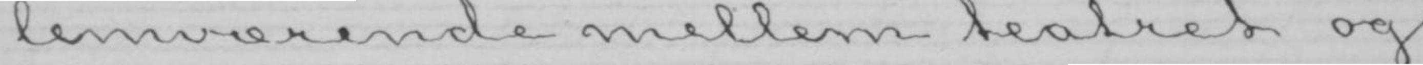lemværende mellem teatret og
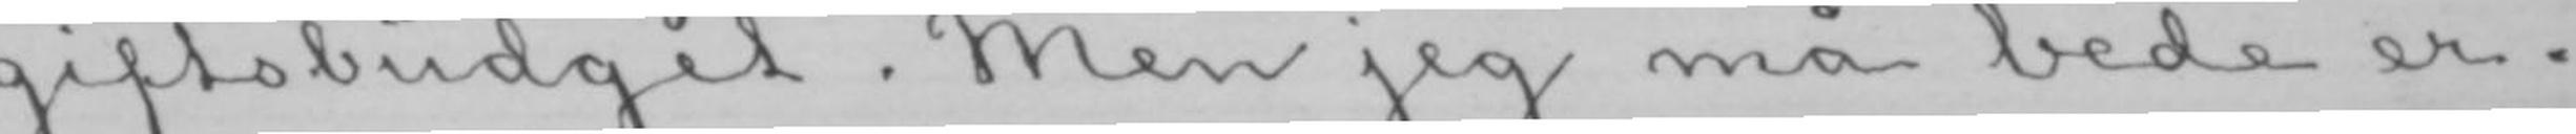giftsbudget. Men jeg må bede er-
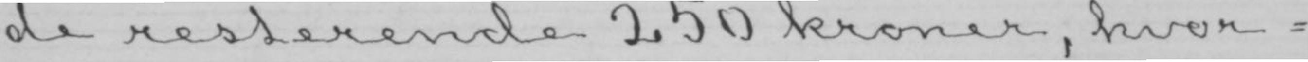de resterende 250 kroner, hvor-
